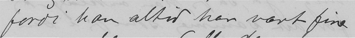fordi han altid har vært fine
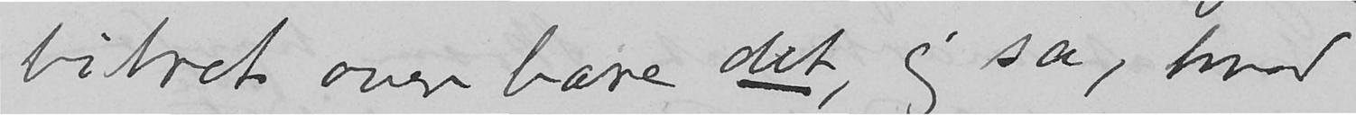bitret over bare det, jeg sa, hvad
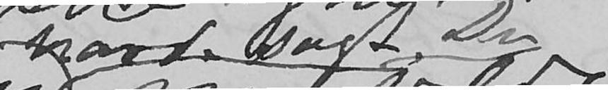havde sagt Du
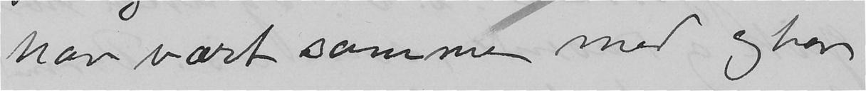han vært samme med og har
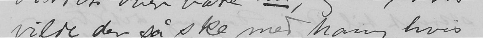vilde der så ske med han, hvis
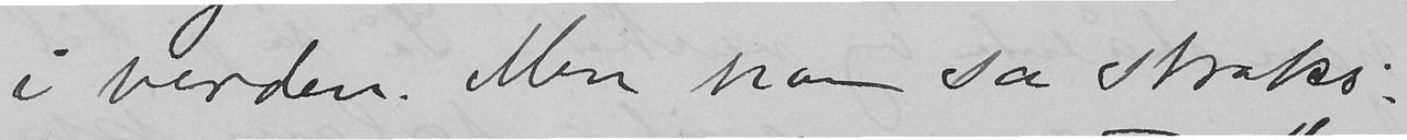i verden. Men han sa straks:
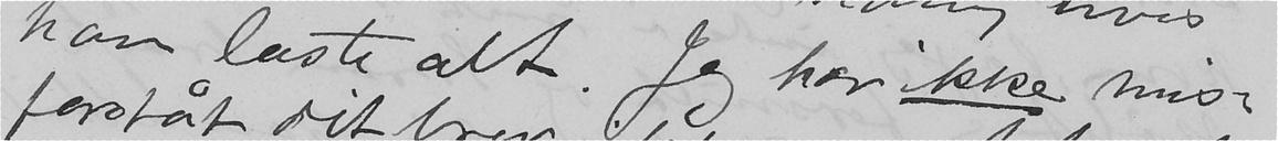han læste alt. Jeg har ikke mis-
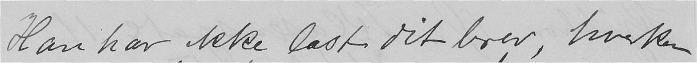Han har ikke læst dit brev, hverken
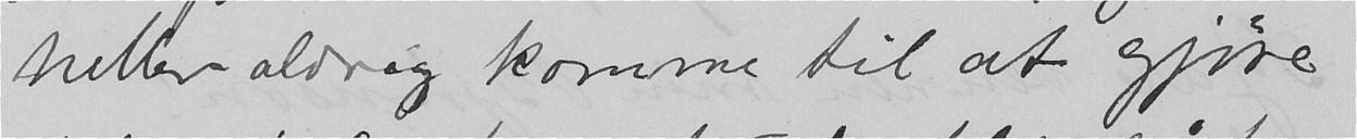heller aldrig komme til at gjøre
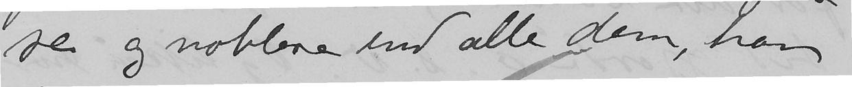re og noblere end alle dem, han
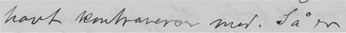havt kontroverser med. Så er
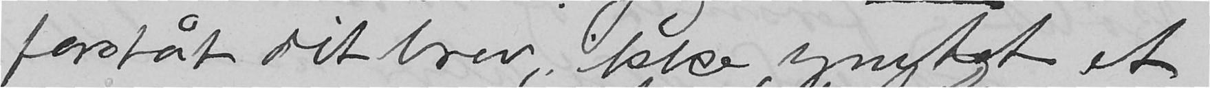forståt dit brev, ikke ymtet et
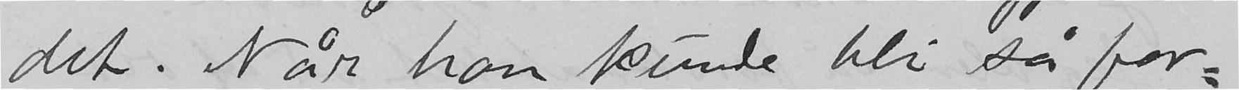det. Når han kunde bli så for-
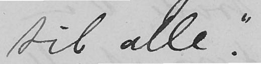til alle."
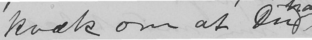kvæk om at Du
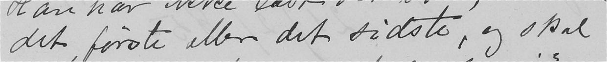det første eller det sidste, og skal
